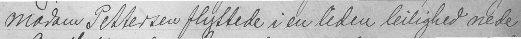madam Pettersen flyttede i en liden leilighed nede
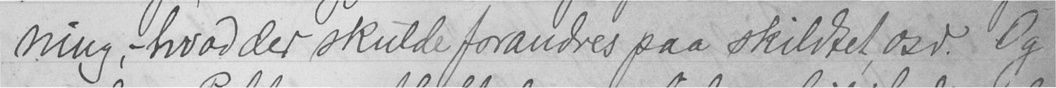ning, - hvad der skulde forandres paa skildtet, osv. Og
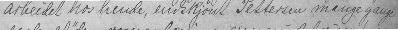arbeidet hos hende, endskjønt Pettersen mange gange
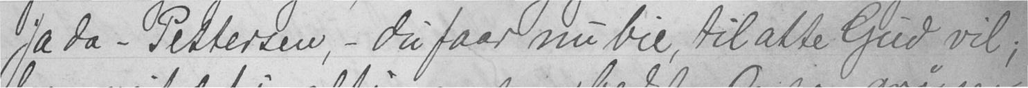Ja da - Pettersen, - du faar nu bie, til atte Gud vil;
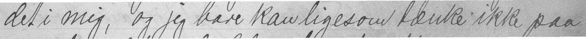det i mig, og jeg bare kan ligesom tænke ikke paa
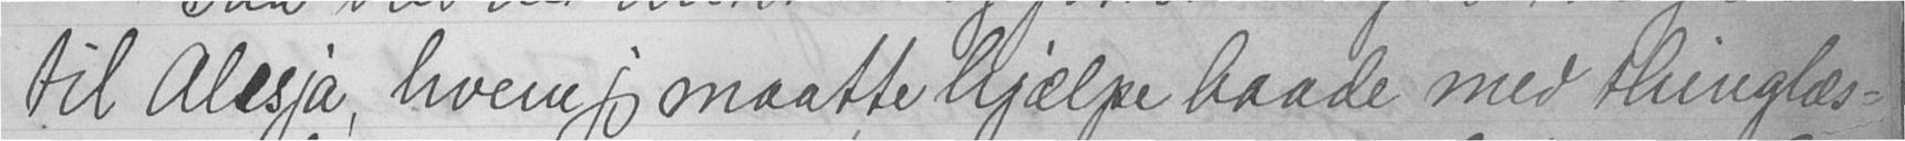til Alesja, hvem jeg maatte hjælpe baade med thinglæs-
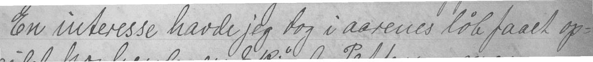En interesse havde jeg dog i aarenes løb faaet op-
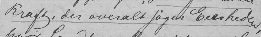Kraft, der overalt søger Eenheden,
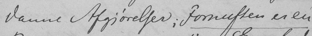danne Afgjørelser; Fornuften er en
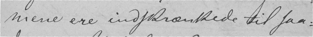mene ere indskrænkede til saa-
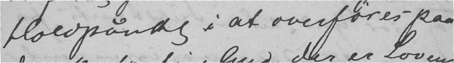Holdepunkt i at overføres paa
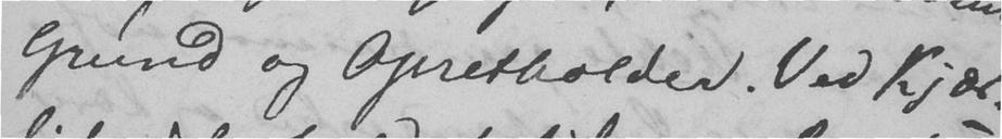Grund og Opretholder. Ved Kjær-
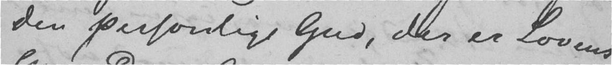den personlige Gud, der er Lovens
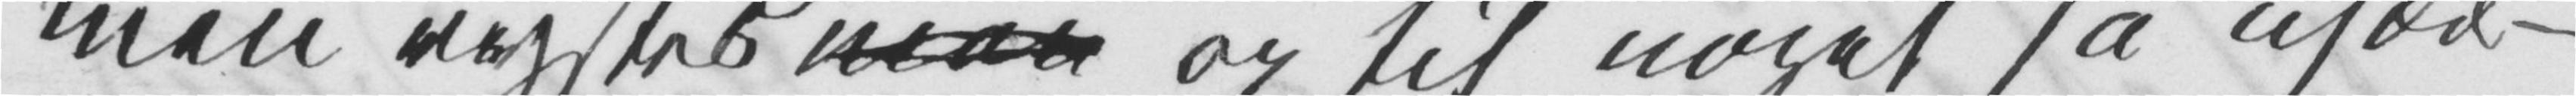man rystes man op til noget så usæd¬
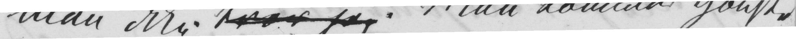man ikke tror jeg. Man kommer ganske
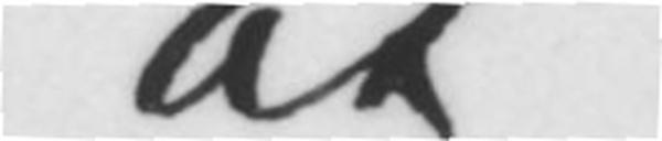at
